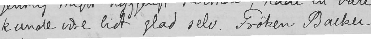kunde være lidt glad selv. Frøken Backer
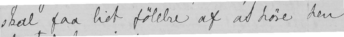skal faa lidt følelse af at høre hen
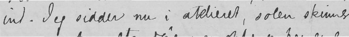ind. Jeg sidder nu i atelieret, solen skinner
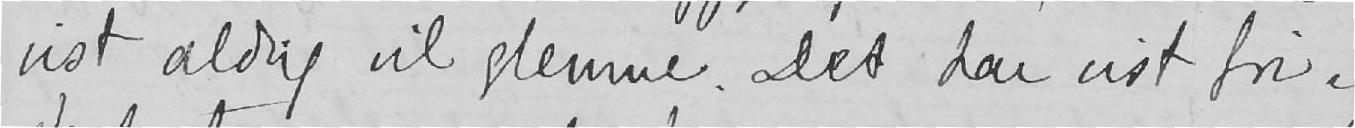vist aldrig vil glemme. Det har vist fris-
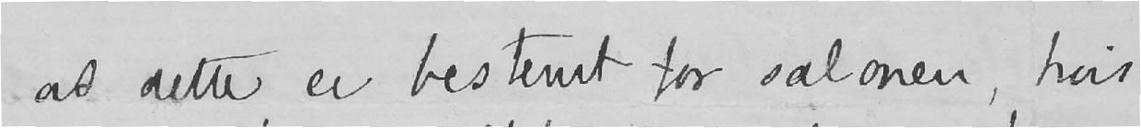at dette er bestemt for salonen, hvis
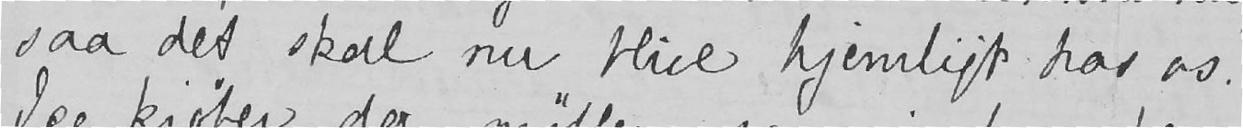saa det skal nu blive hjemligt hos os.
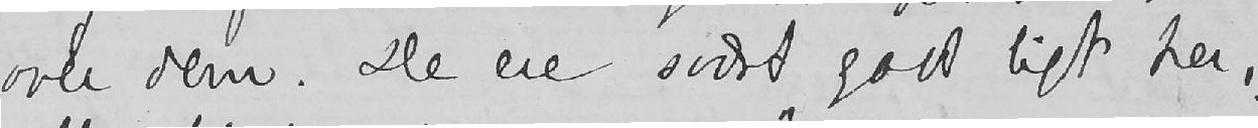over dem. De ere svært godt ligt her,
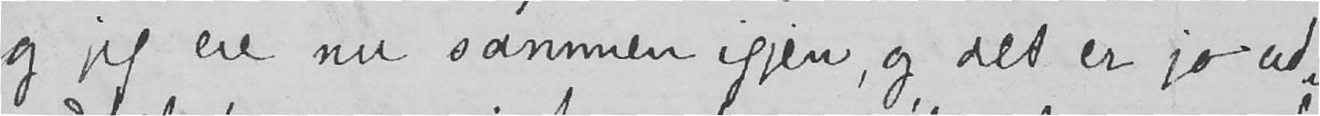og jeg ere nu sammen igjen, og det er jo ud-
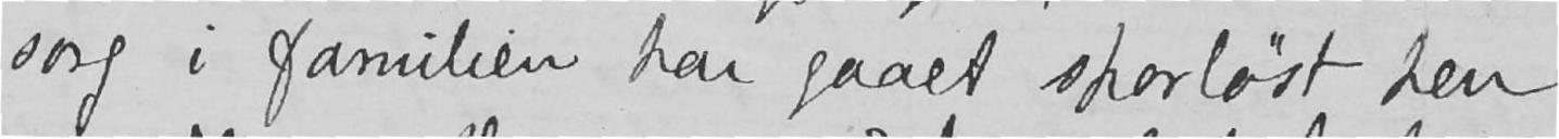sorg i familien har gaaet sporløst hen
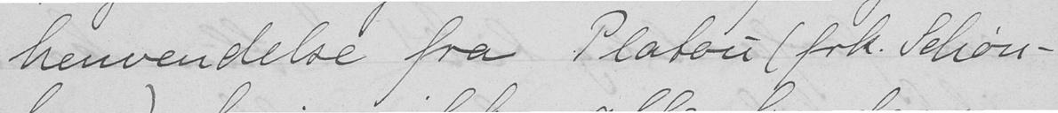henvendelse fra Platou (frk. Schøn-
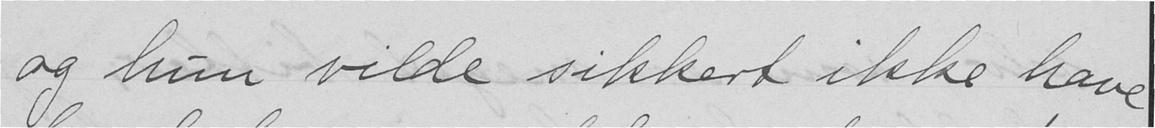og hun vilde sikkert ikke have
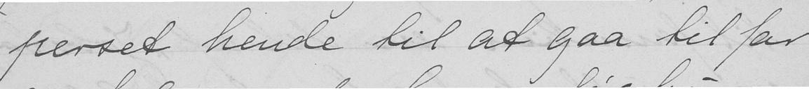perset hende til at gaa til far
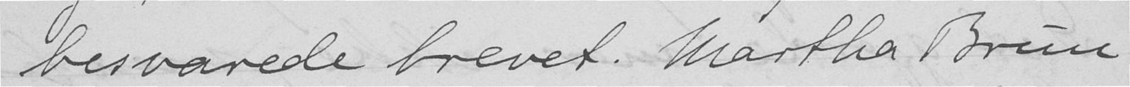besvarede brevet. Martha Brun
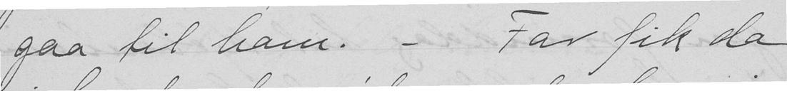gaa til ham. - Far fik da
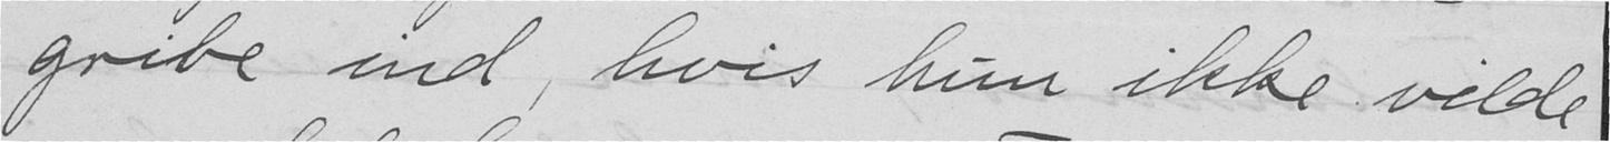gribe ind, hvis hun ikke vilde
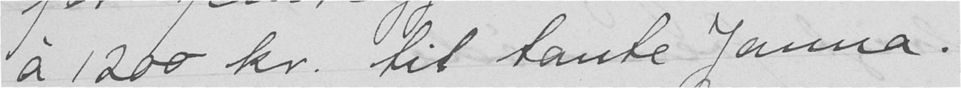à 1200 kr. til tante Janna.
